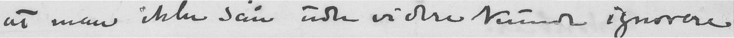at man ikke sån uden videre kunde ignorere
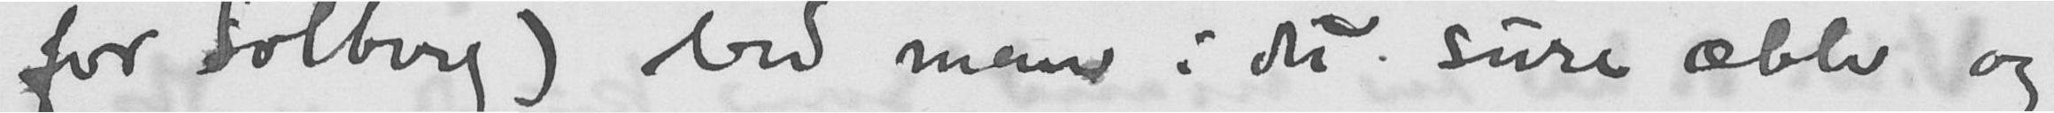for Solberg) bed man i det sure æble og
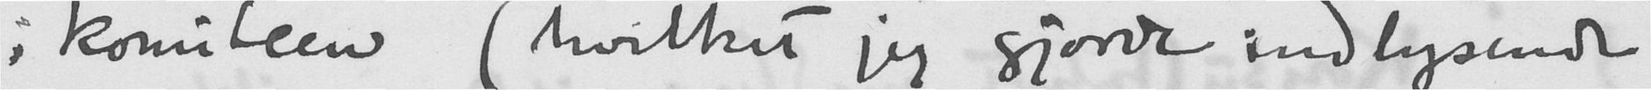i komiteen (hvilket jeg gjorde indlysende
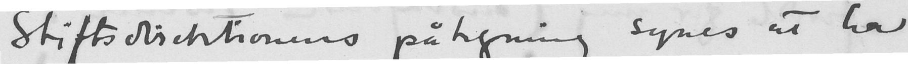Stiftsdirektionens påtegning synes at ha
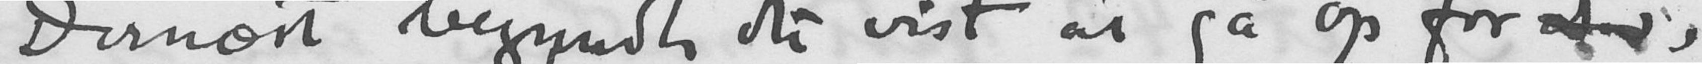Dernæst begyndte det vist at gå op for dem,
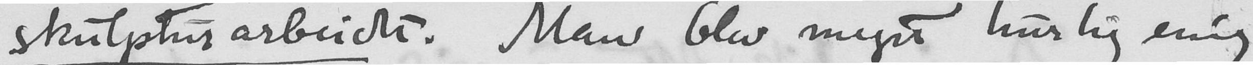skulpturarbeidet. Man blev meget hurtig enig
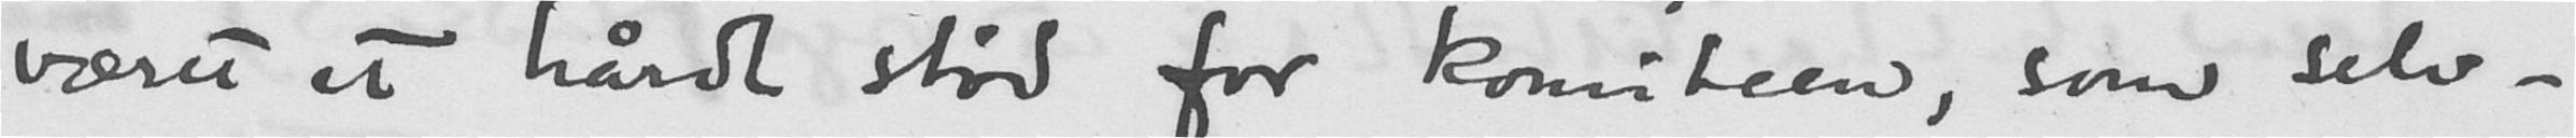været et hårdt stød for komiteen, som selv-
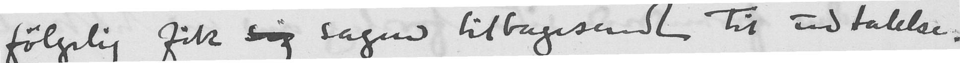følgelig fik sig sagen tilbagesendt til udtalelse.
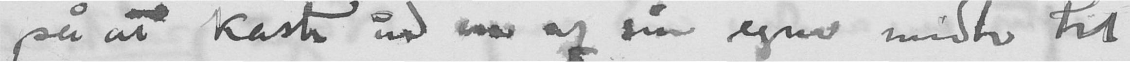på at kaste ud en af sin egen midte til
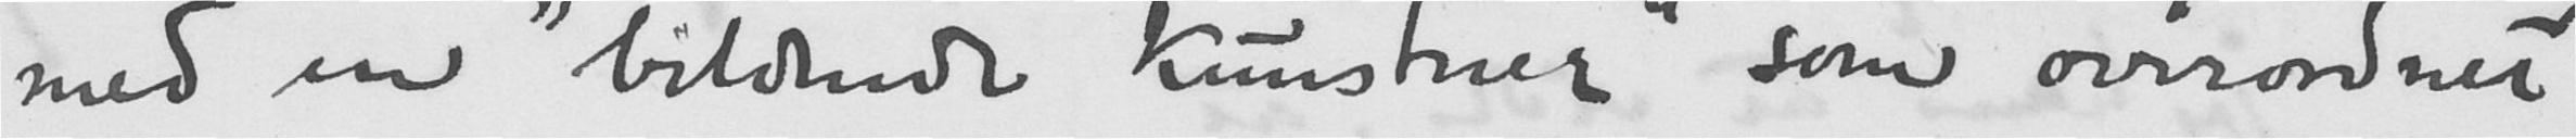med en "bildende kunstner" som overordnet
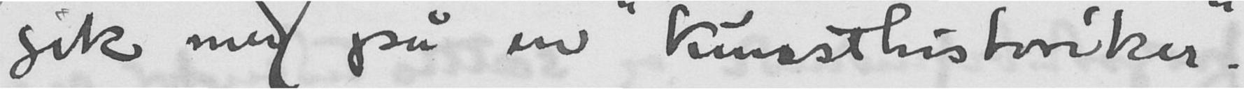gik med på en "kunsthistoriker".
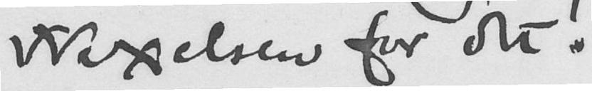Wexelsen for det!
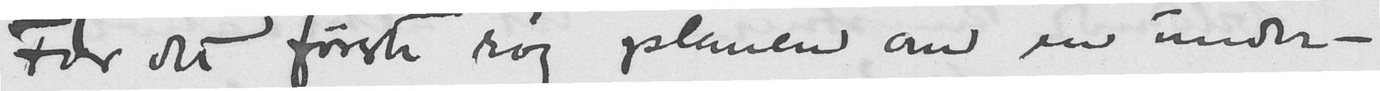For det første røg planen om en under-
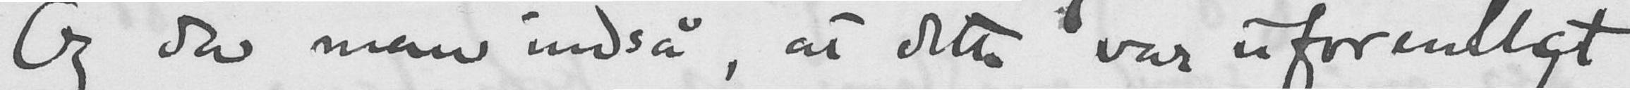Og da man indså, at dette var uforenligt
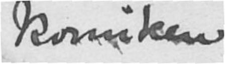komiteen
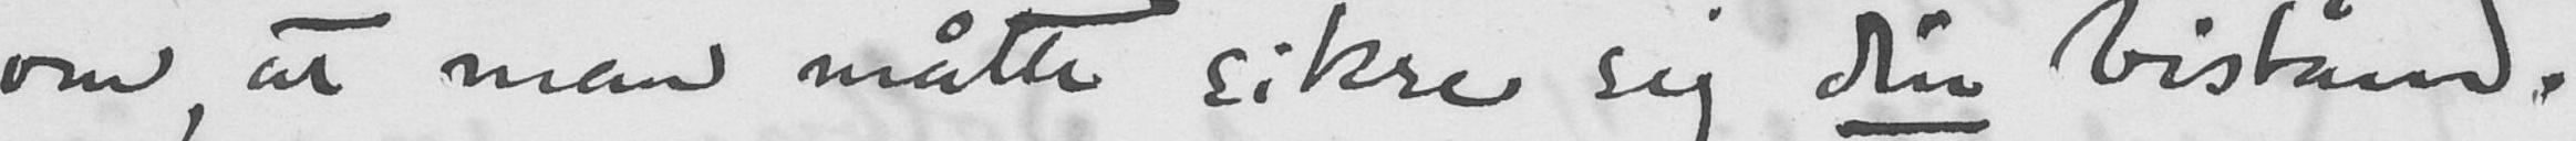om, at man måtte sikre sig din bistand.
